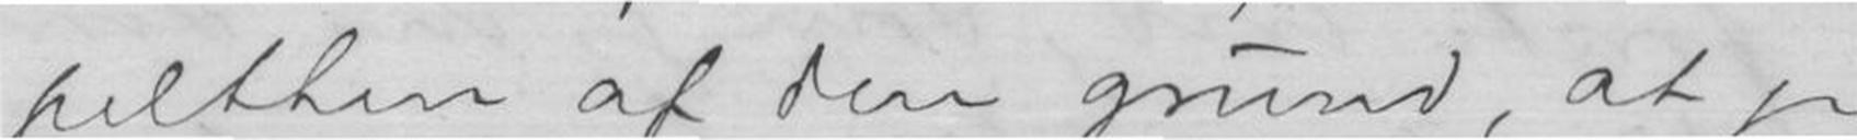pelthen af den grund, at jeg
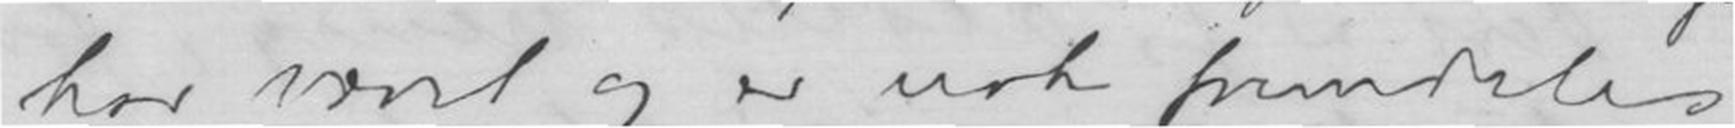har vært og er nok fremdeles
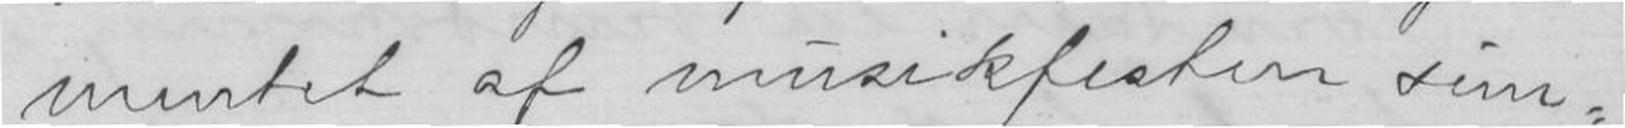mentet af musikfesten sim-
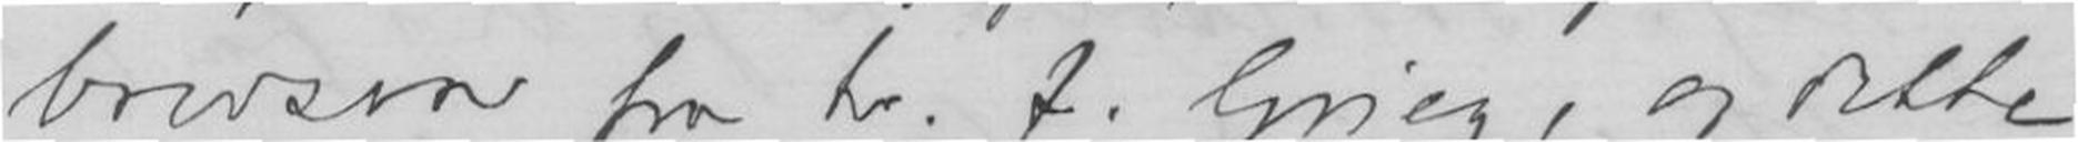brevsvar fra hr. J. Grieg, og dette
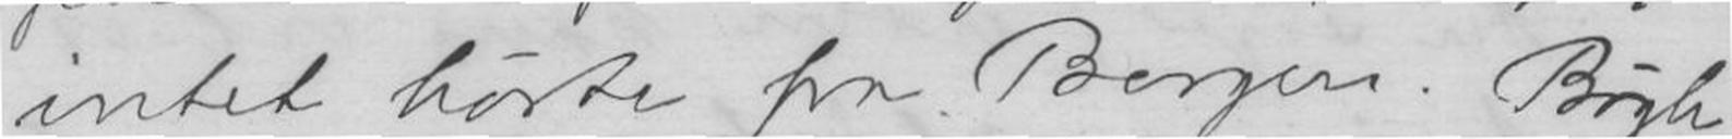intet hørte fra Bergen. Bøgh
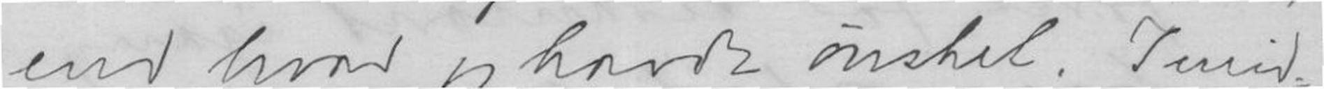end hvad jeg havde ønsket. Imid
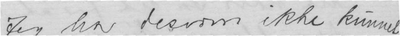Jeg har desværre ikke kunnet
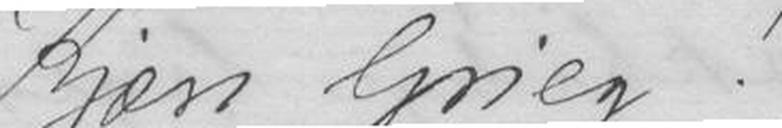Kjære Grieg!
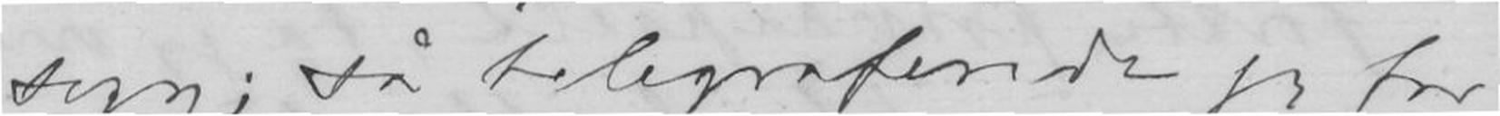syg; så telegraferede jeg for
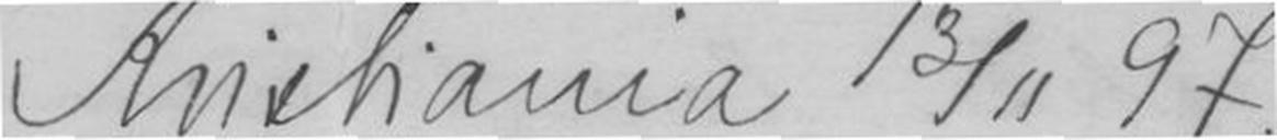Kristiania 13/11 97
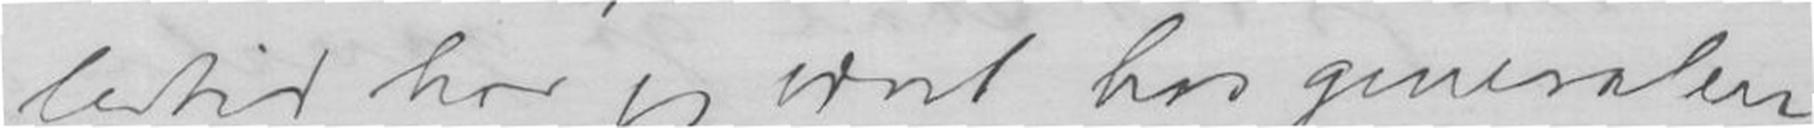lertid har jeg vært hos generalen
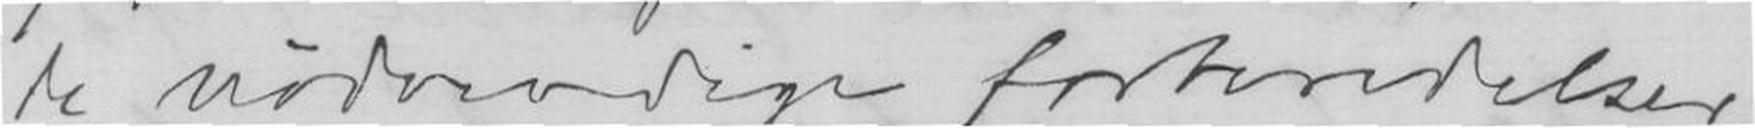de nødvendige forberedelser
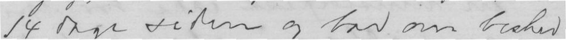14 dage siden og bad om besked
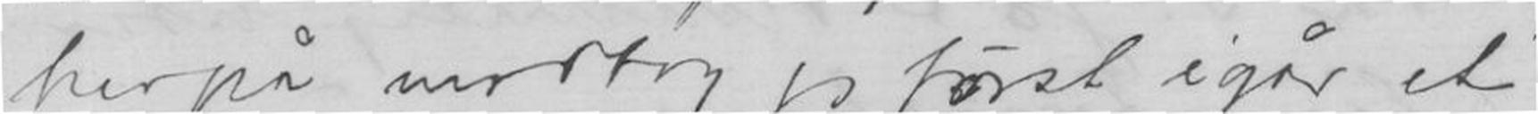herpå modtog jeg først igår et
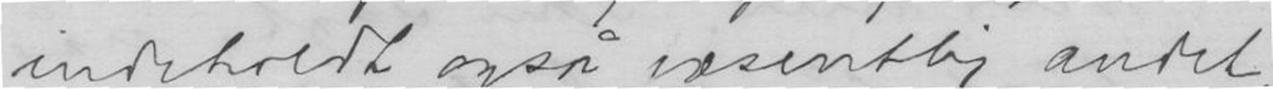indeholdt også væsentlig andet
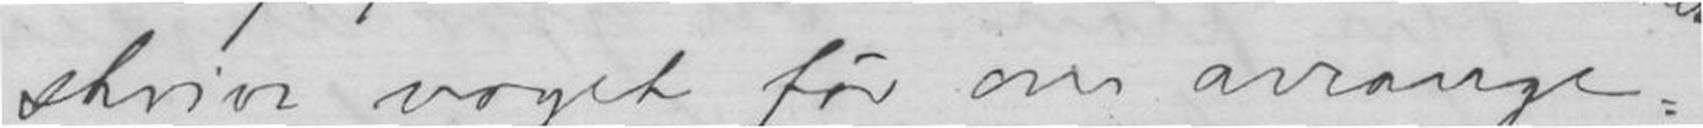skrive noget før om arrange-
